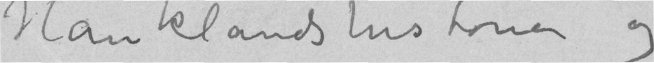Hauklandshistorien og
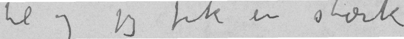til og jeg fik en stærk
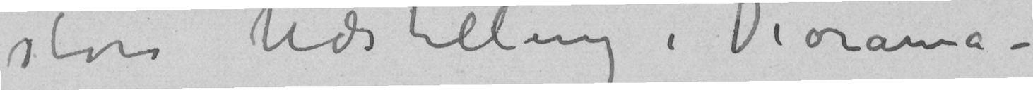store Udstilling i Diorama-
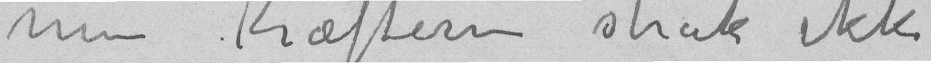men Kræfterne strak ikke
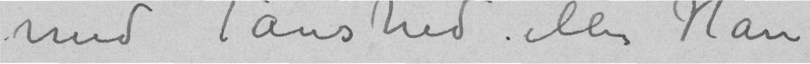med Taushed eller Hån
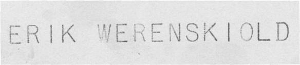ERIK WERENSKIOLD
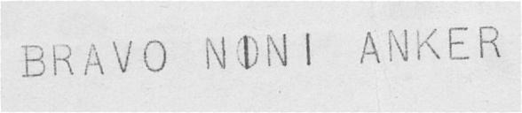BRAVO NINI ANKER
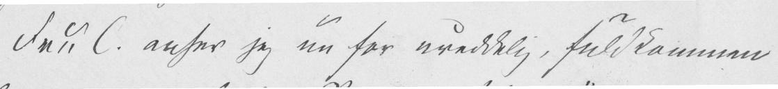Fru C. anser jeg nu for ureddelig, fuldkommen
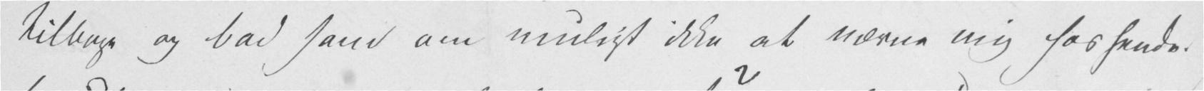tilbage og bad ham om muligt ikke at nævne mig hos hende.
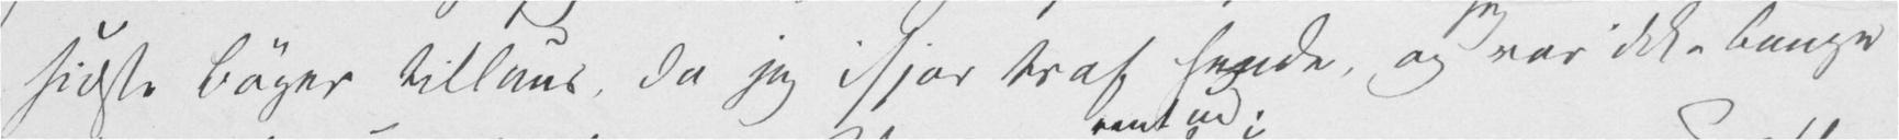sidste Bøger tillåns, da jeg ifjor traf hende, og var ikke bange
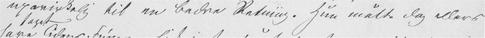upåvirkelig til en bedre Retning. Hun måtte dog ellers
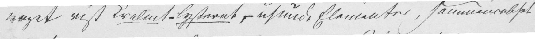noget vist kvalmt – lysteret – usunde Elementer, sammenrabset
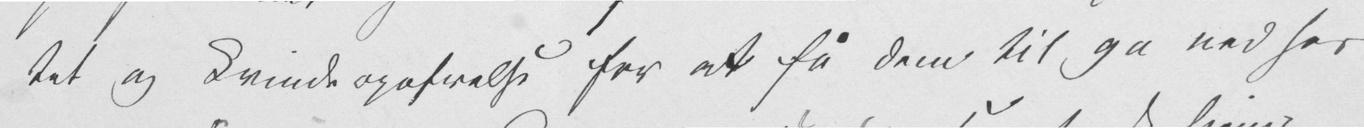tet og Kvindeopofrelse for at få dem til ga ned hos
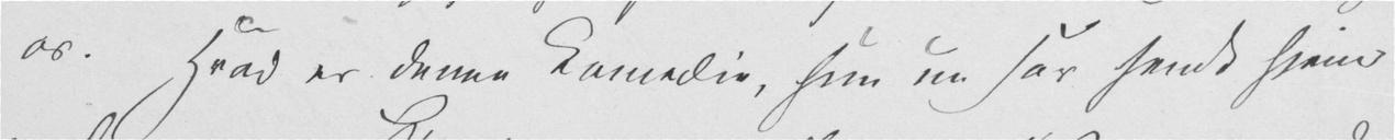os. Hvad er denne Komedie, hun nu har sendt hjem
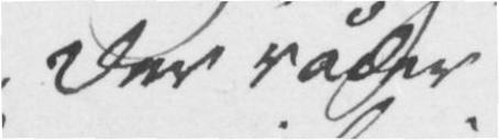Der råder
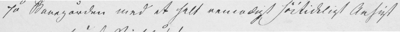på Banegården med et helt vemodigt høitideligt Ansigt
